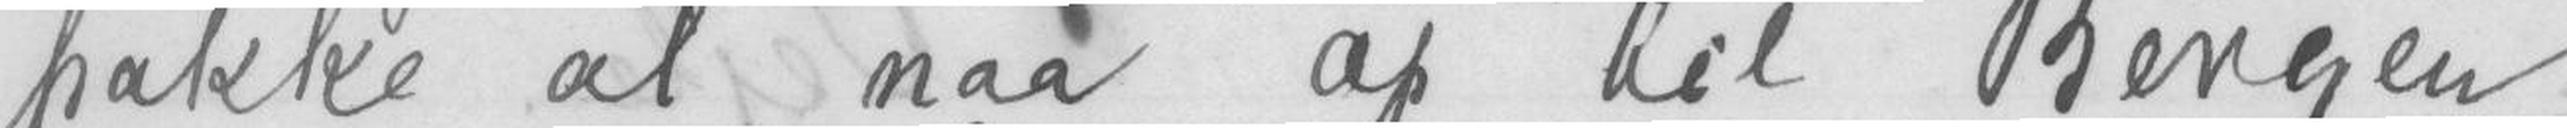pakke al naa op til Bergen
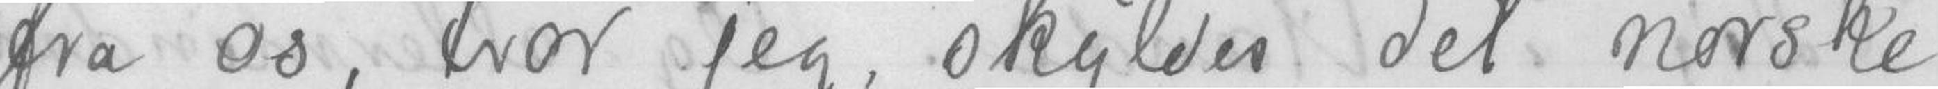fra os, tror jeg, skyldens det norske
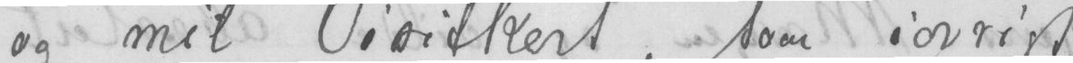og mit visitkent, som iøvrigt
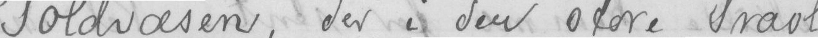der i den store Travl-
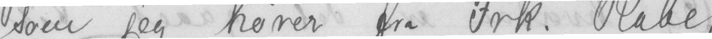som jeg hører fra Frk. Rable,
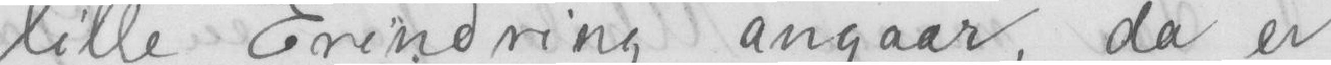"lille Erindring" angaar, da er
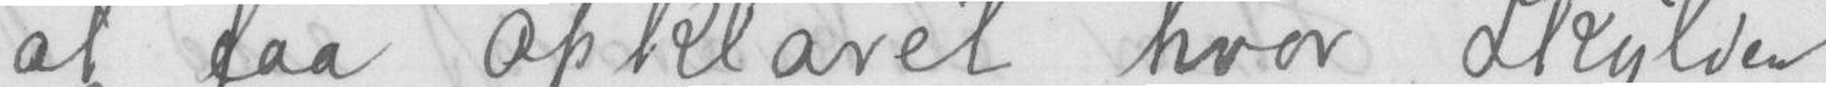at faa opklaret hvor Skylden
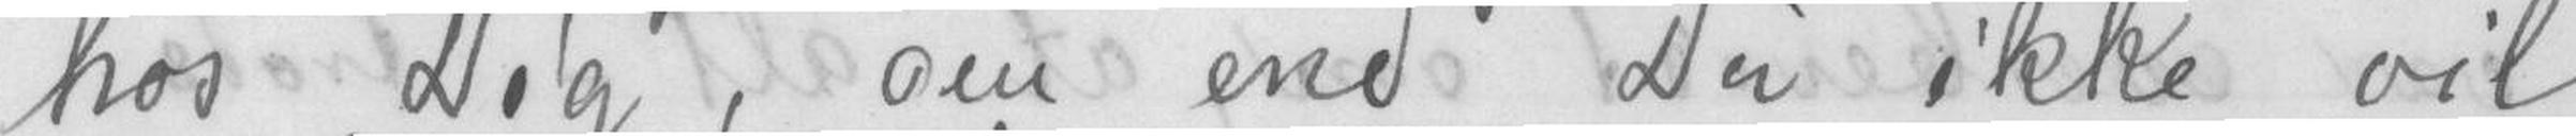hos Dig, om end Du ikke vil
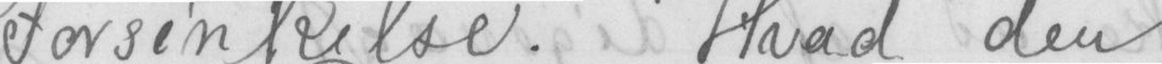Forsinkelse. Hvad den
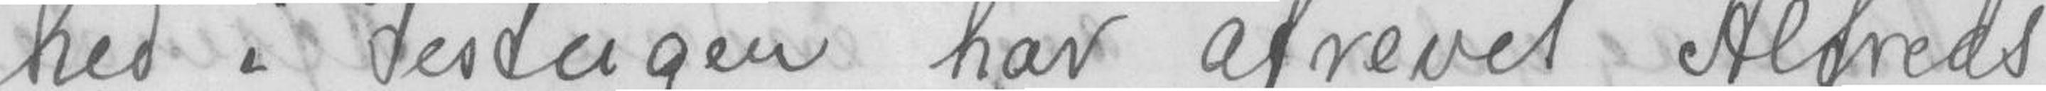hed i Festugen har afrevel Alfreds
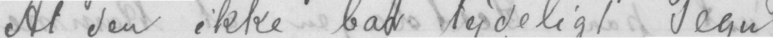At den ikke bar tydeligt Tegn
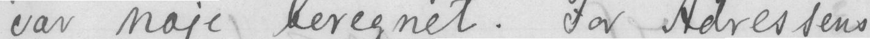var nøje beregnet. For Adressens
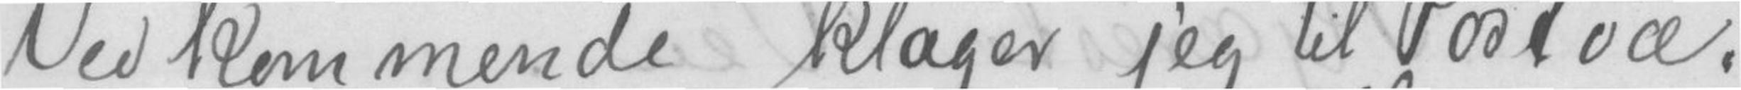Vedkommende klager jeg til Postvæ-
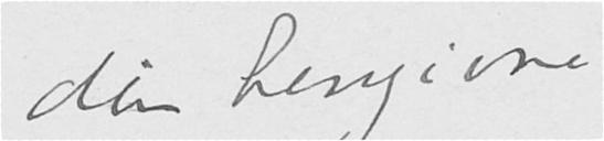din hengivne
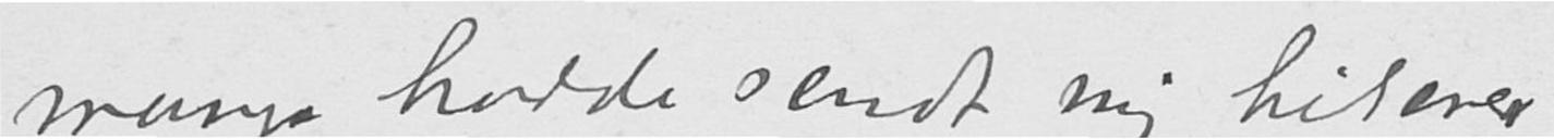mange hadde sendt mig hilsener
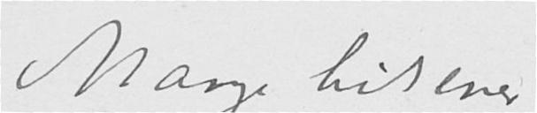Mange hilsener
